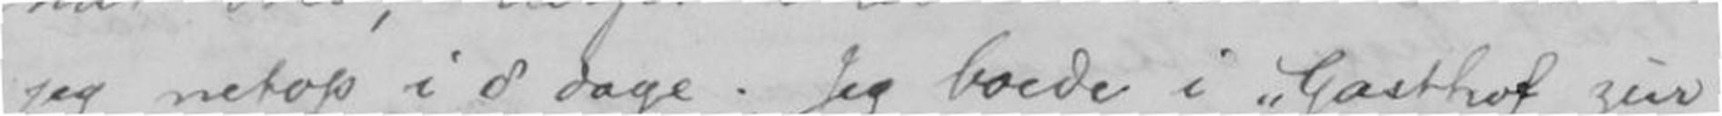jeg netop i 8 dage. Jeg boede i "Gasthof zur
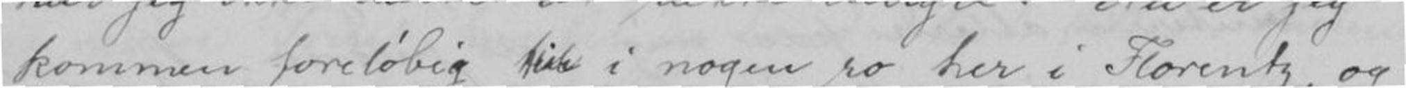kommen føreløbig i nogen ro her i Florentz, og
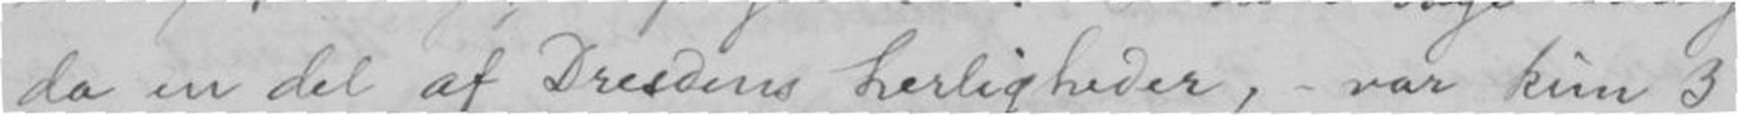jeg da en del af Dresdens herligheder, - var kun 3
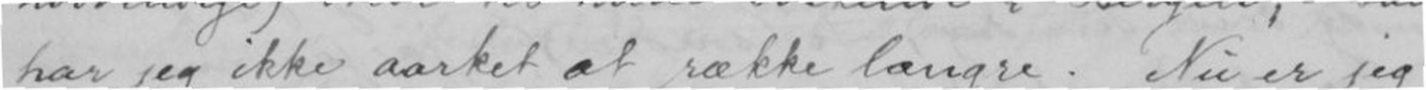har jeg ikke aarket at række længre. Nu er jeg
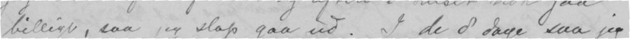billige, saa jeg slap gaa ud. I de 8 dage saa
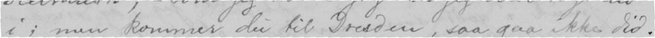i; men kommer du til Dresden, saa gaa ikke did.
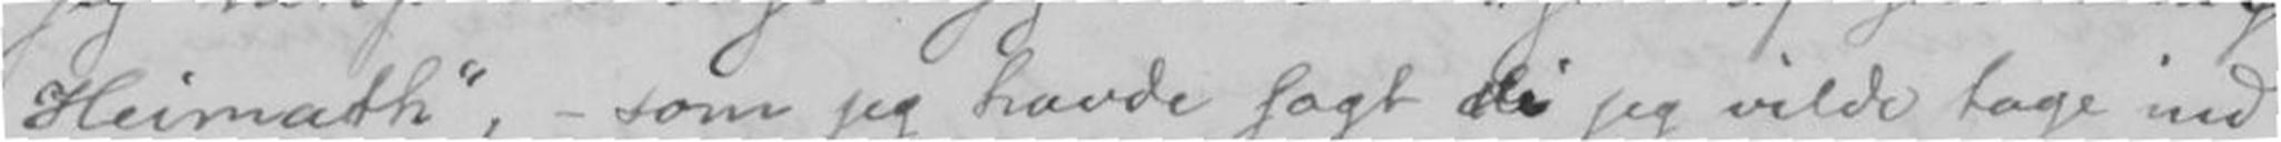Heimath", - som jeg havde sagt jeg vilde tage ind
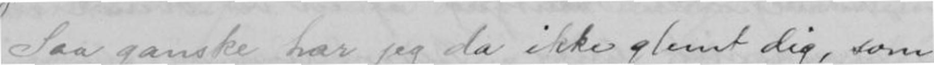Saa ganske har jeg da ikke glemt dig, som
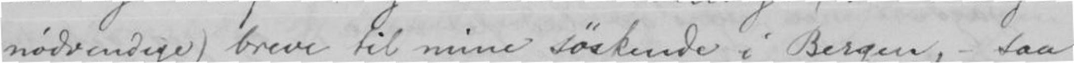nødvendige) breve til mine søskende i Bergen, - saa
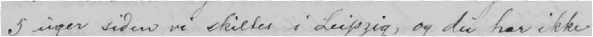5 uger siden vi skiltes i Leipzig, og du har ikke
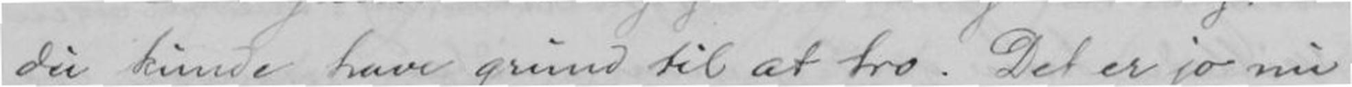du kunne have grund til at tro. Det er jo nu
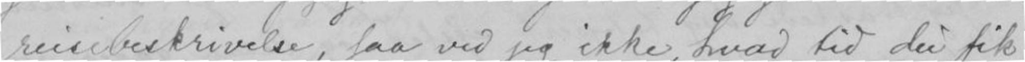reisebeskrivelse, saa ved jeg ikke hvad tid du fik
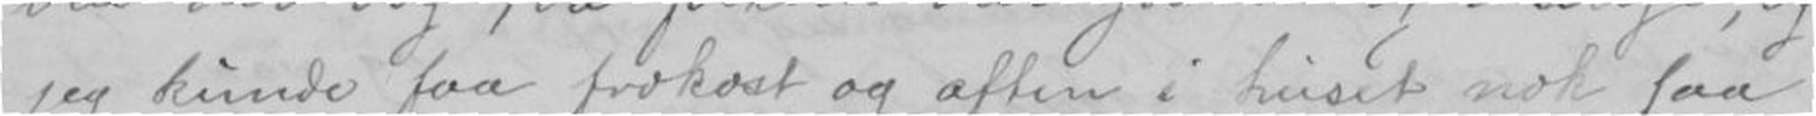jeg kunde faa frokost og aften i huset nok saa
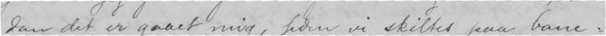dan det er gaaet mig, siden vi skiltes paa bane-
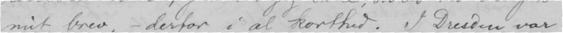mit brev, - derfor i al korthed. I Dresden var
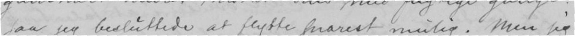saa jeg besluttede at flytte snarest mulig. Men jeg
